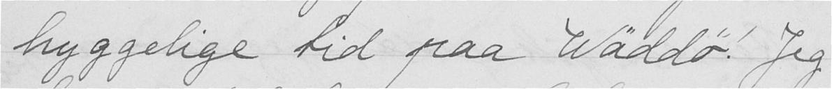hyggelige tid paa Wäddö! Jeg
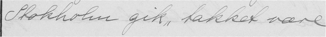Stokholm gik, takket være
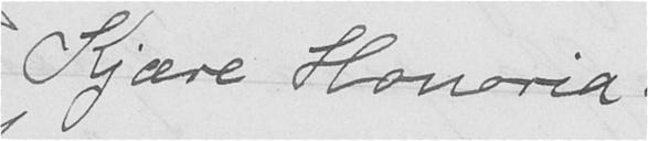Kjære Honoria!
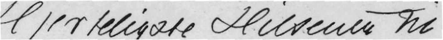Hjerteliste Hilsener til
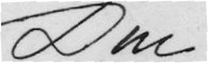Din
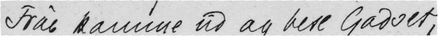Frue komme ud og bere Godvet,
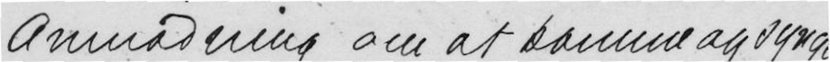anmodning om at komme og synge
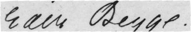Eder Begge.
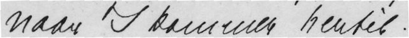naar I kommer hertil
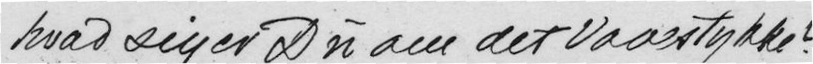hvad siger Du om det vaarstykke?
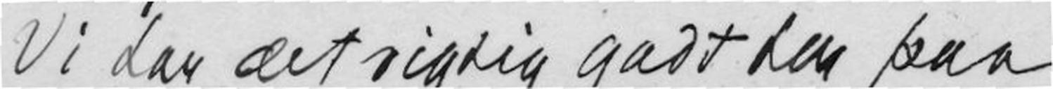Vi har det rigtig godt her paa
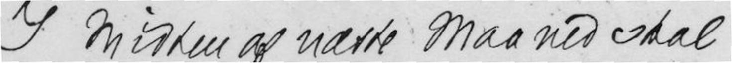I Midten af næste Maaned skal
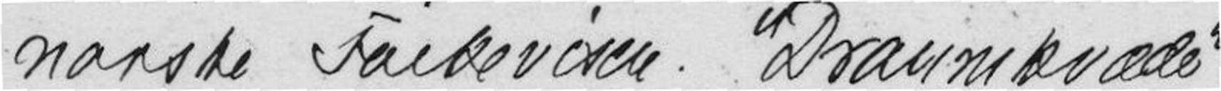norske Folkeviser "Draumkvæde"
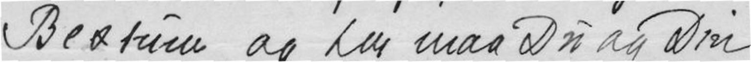Bestum og maa Du og Din
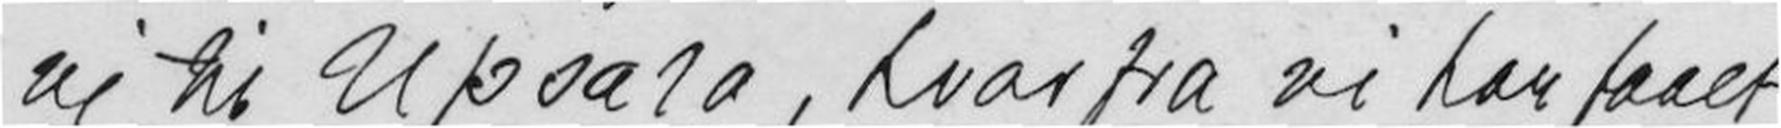vi til Upsalo, hvorfra vi har faaet
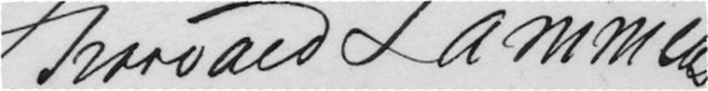Thorvald Lammers
